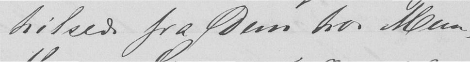hilsede fra Dem hos Mun-
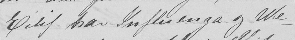Eilif har Influenza og We-
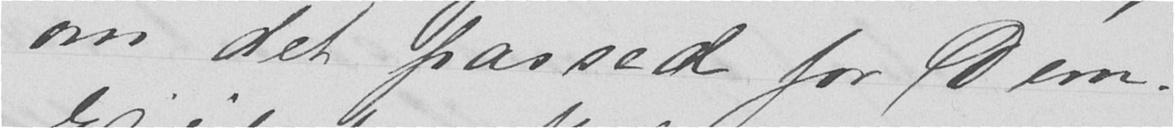om det passed for Dem.
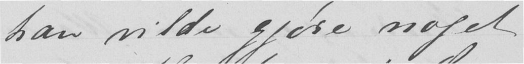han vilde gjøre noget
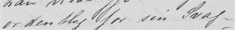ordentlig for sin Svag-
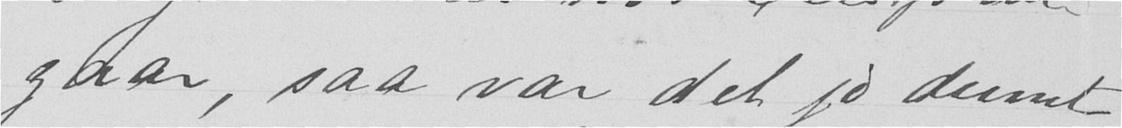gaar, saa var det jo dumt
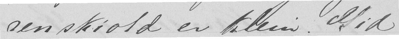renskiold er klein. Gid
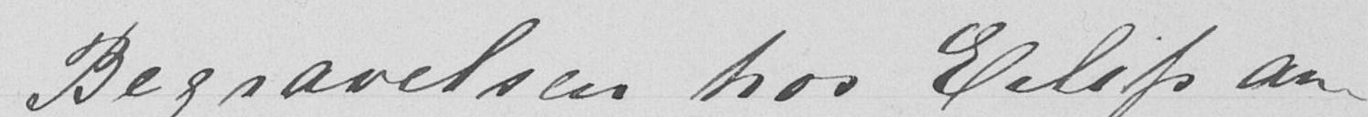Begravelsen hos Eilifs an-
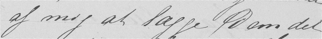af mig at lægge Dem det
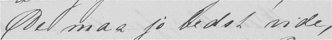De maa jo bedst vide,
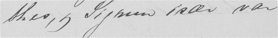thes, jeg Sigrun især var
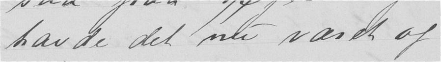havde det nu været og
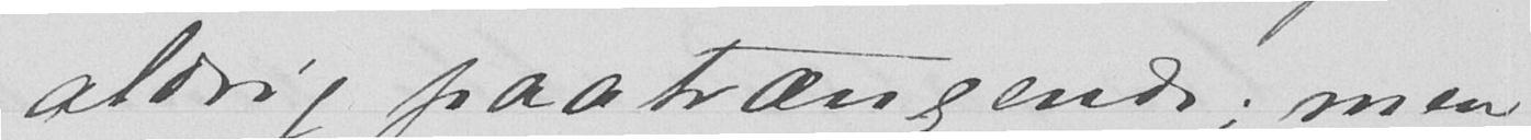aldrig paatrængende; men
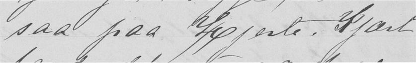saa paa Hjerte. Kjært
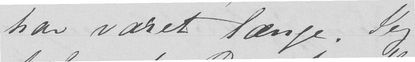har varet længe. Jeg
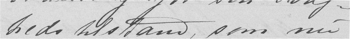heds tilstand, som nu
